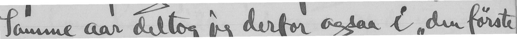Samme aar deltog jeg derfor ogsaa i "den første"
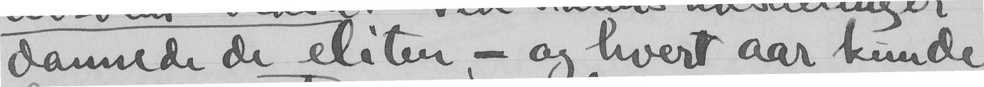dannede de eliten - og hvert aar kunde
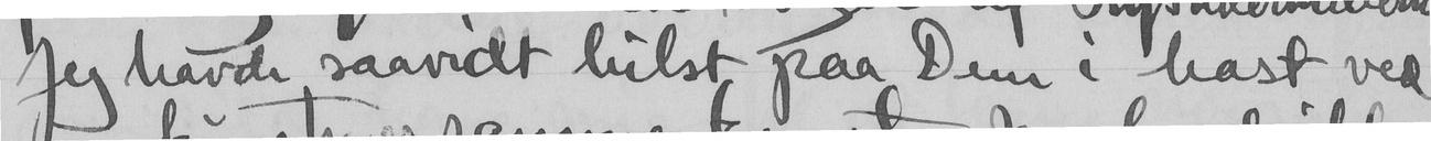Jeg havde saavidt hilst paa Dem i hast ved
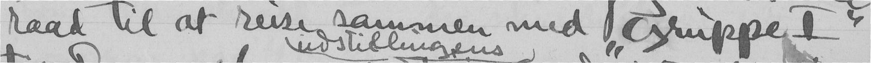raad til at reise sammen med "Gruppe I"
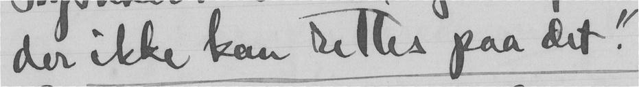der ikke kan rettes paa det".
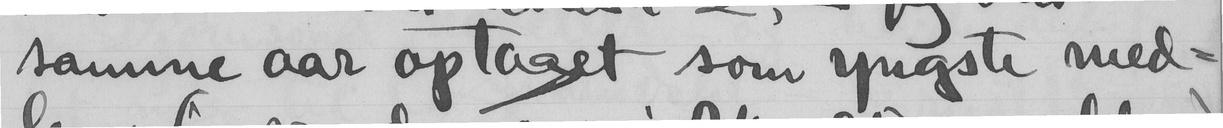samme aar optaget som yngste med-
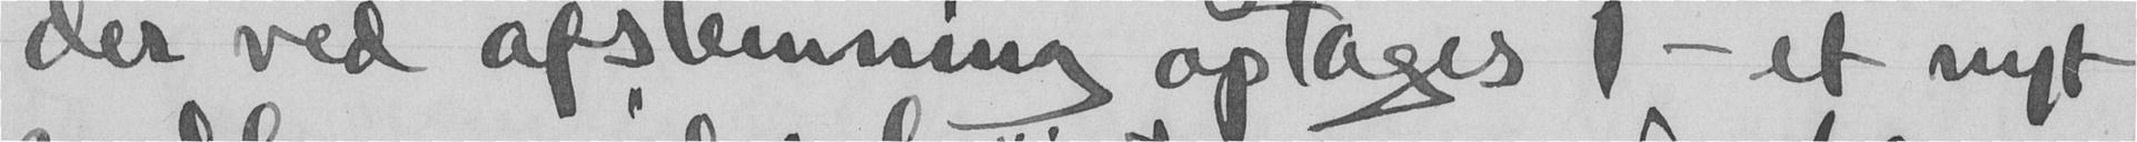der ved afstemning optages 1 - et nyt
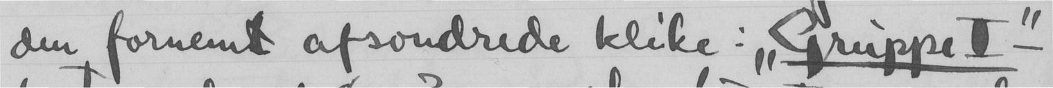den fornemt afsondrede klike: "Gruppe I"-
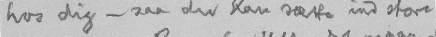hos dig - saa du kan sætte ind store
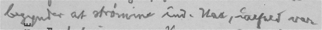begynder at strømme ind. Naa, ialfald var
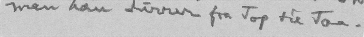men han dirrer fra Top til Taa.
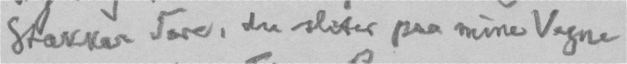Stakkar Tore, du sliter paa mine Vegne
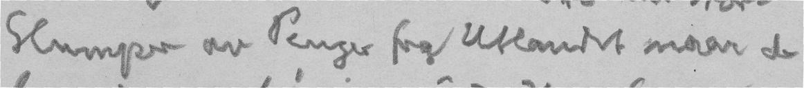Slumper av Penger fra Utlandet naar de
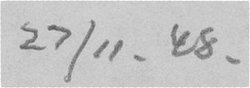27/11. 48.
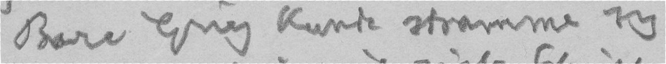Bare Grieg kunde stramme sig
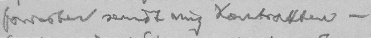forresten sendt mig Kontrakten -
